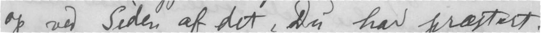op ved Siden af det, Du har præstert
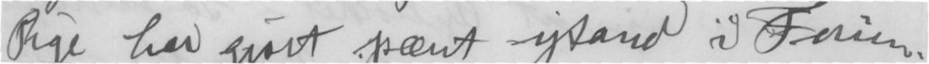Pige har gjort pænt istand i Ferien.
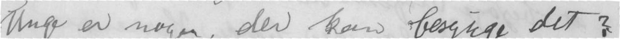Unge er nogen, der kan besynge det?
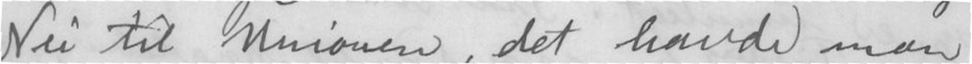Nei til Unionen, det havde man
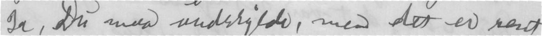Ja, Du maa undskylde, men det er rent
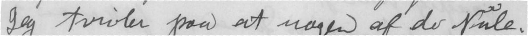Jeg tvivler paa at nogen af de Nule-
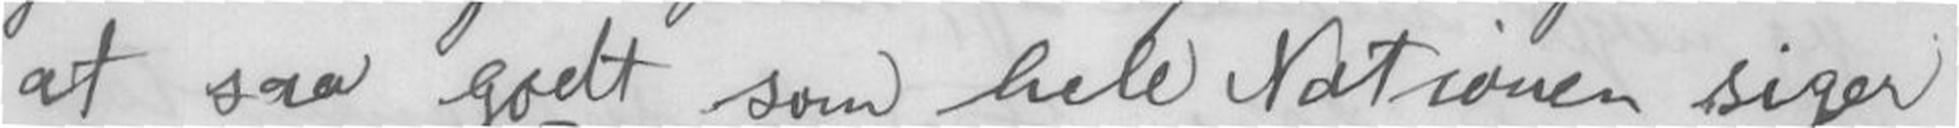at saa godt som hele Nationen siger
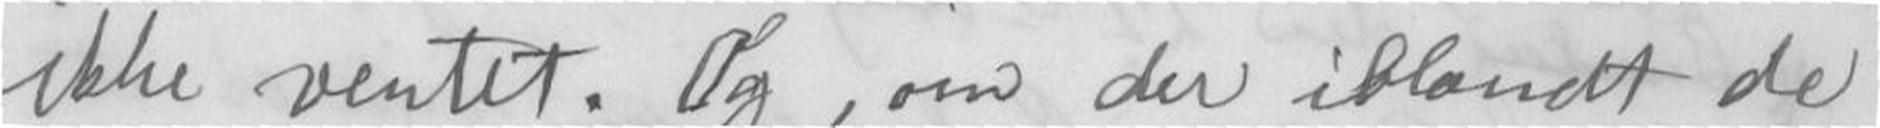ikke ventet. Og, om der iblandt de
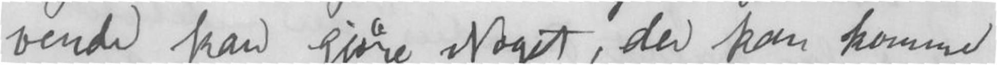vende kan gjøre Noget, der kan komme
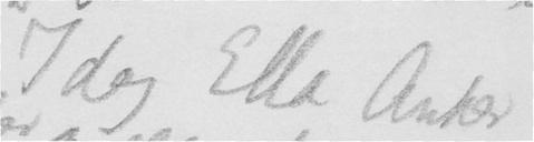Idag Ella Anker
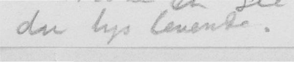du lys levende.
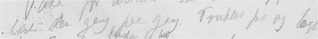læst den gang på gang. Trukket for og læst
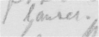landet.
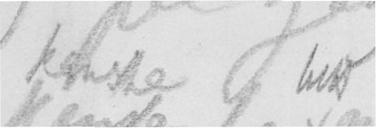fnste. Og hikt
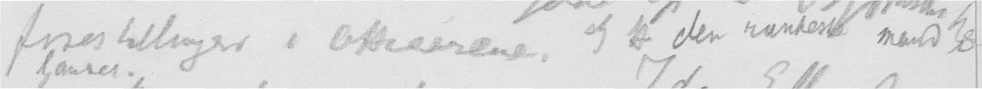forestillinger i Okeaaræne. Og den rankeste mand i
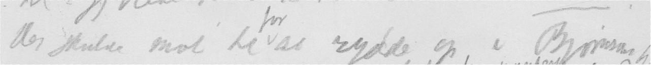Det skulde mot til for at rydde op i Bjørnsons
